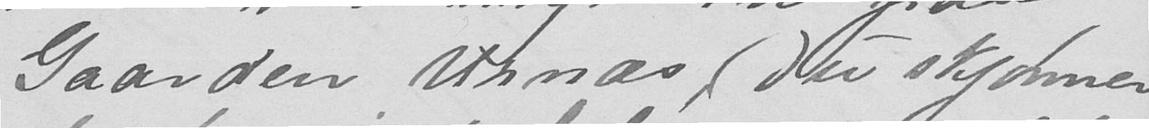Gaarden Urnæs, Du skjønner
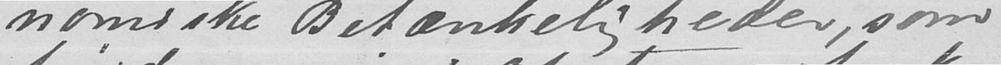nomiske Betænkeligheder som
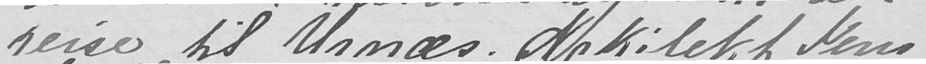reise til Urnæs. Arkitekt Jens
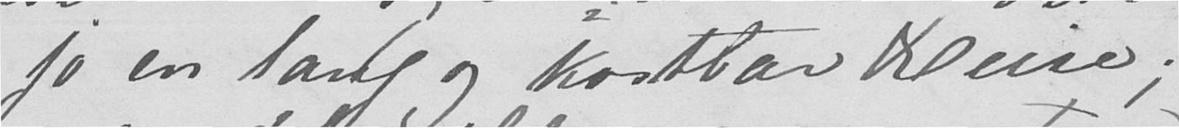jo en lang og kostbar Reise;
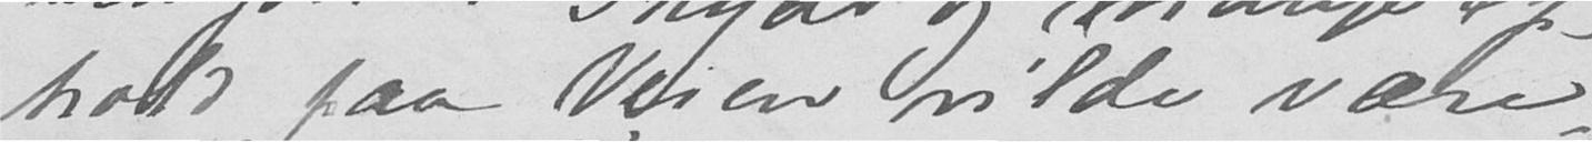hold paa Veien vilde være
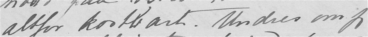altfor kostbart. Undres om jeg
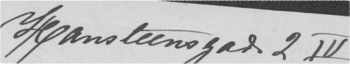Hansteensgade 2 IV
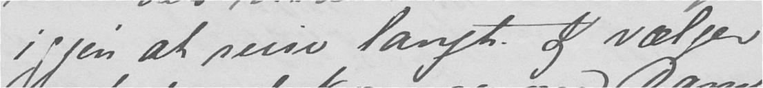igjen at reise langt. Jeg vælger
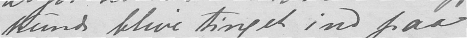kunde blive tinget ind paa
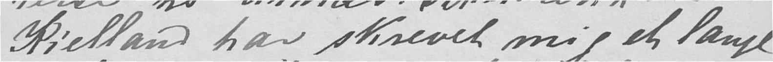Kielland har skrevet mig et langt
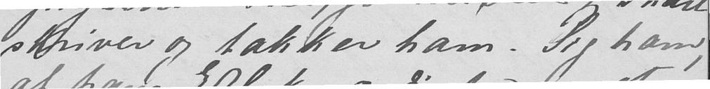skriver og takker ham. Sig ham,
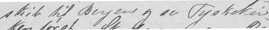skib til Bergen og se Tyskekir-
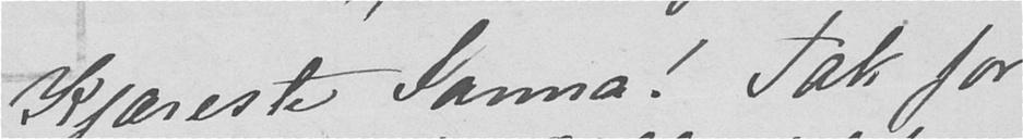Kjæreste Janna! Tak for
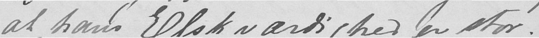at hans Elskværdighed er stor.
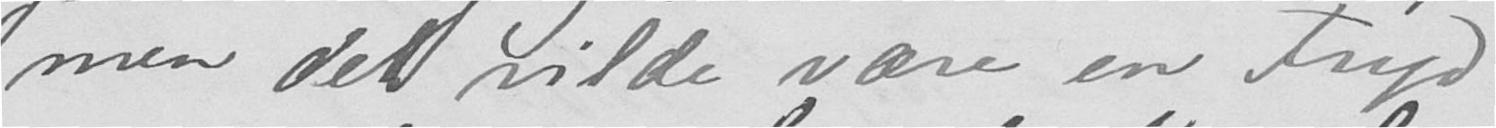men det vilde være en Fryd
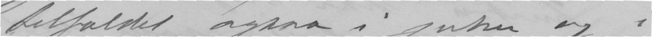tilfældet ogsaa i julen og i
